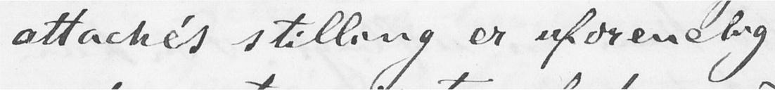attachés stilling er uforenelig
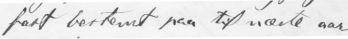fast bestemt paa til næste aar
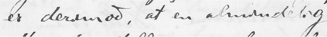er derimod, at en almindelig
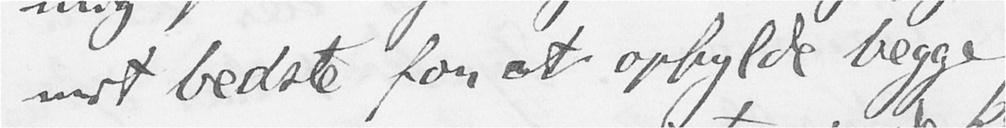mit bedste for at opfylde begge
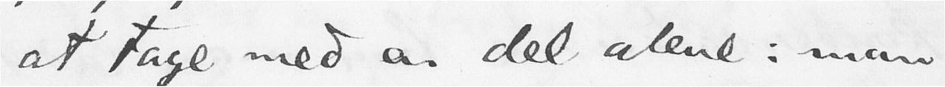at tage med en del alene: man
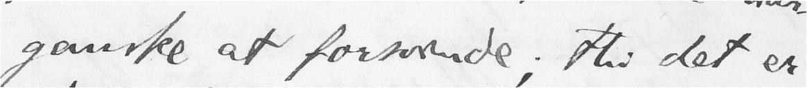ganske at forsvinde; thi det er
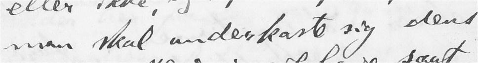man skal underkaste sig dens
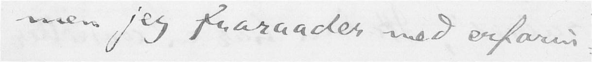men jeg fraraader med erfarin-
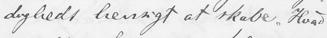digheds hensigt at skabe. Hvad
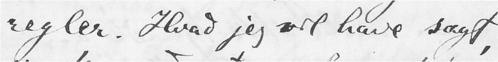regler. Hvad jeg vil have sagt,
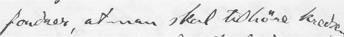fordrer, at man skal tilhøre kredsen
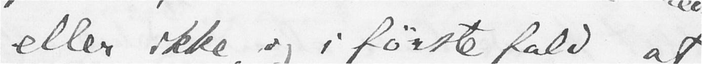eller ikke, og i første fald, at
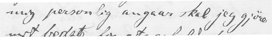mig personlig angaar skal jeg gjøre
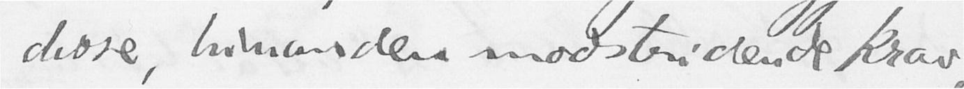disse, hinanden modstridende krav;
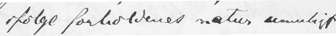ifølge forholdenes natur umuligt
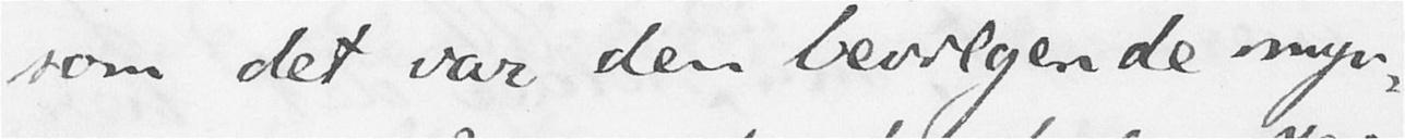som det var den bevilgende myn-
